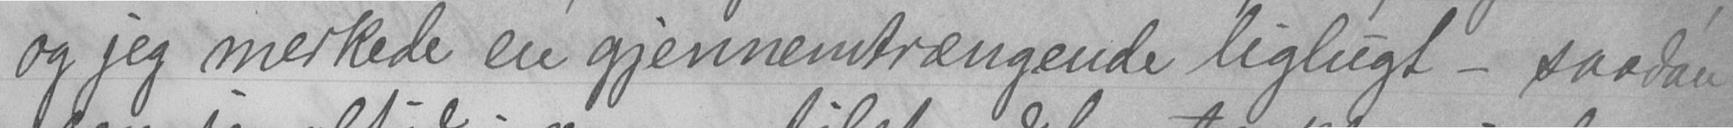og jeg merkede en gjennemtrængende liglugt - saadan
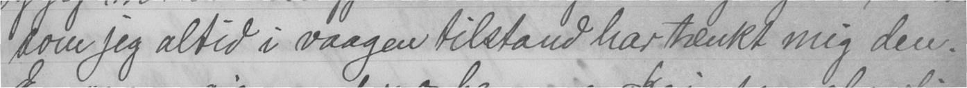som jeg altid i vaagen tilstand har thenkt mig den.
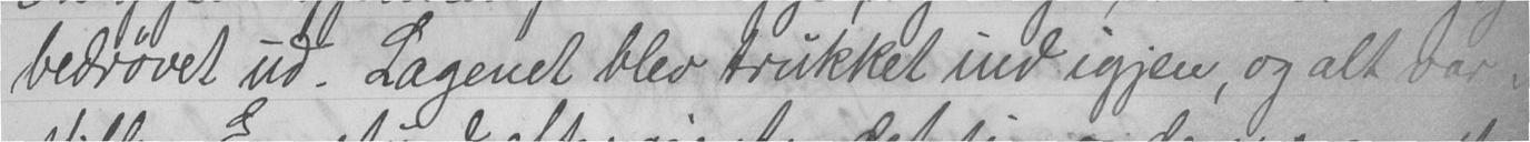bedrøvet ud. Lagenet blev trukket ind igjen, og alt var
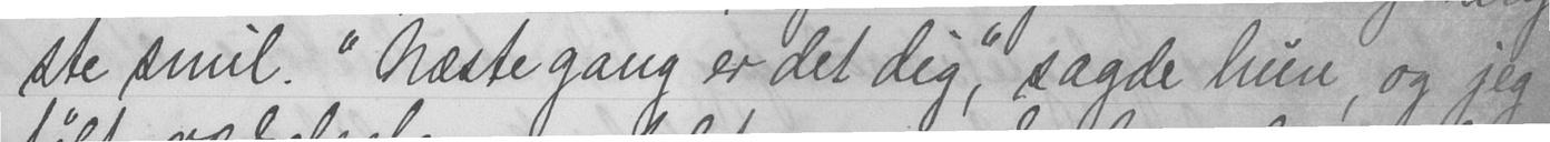ste smil. "Næste gang er det dig", sagde hun, og jeg
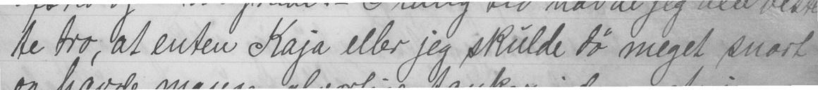te tro at enten Kaja eller jeg skulde dø meget snart
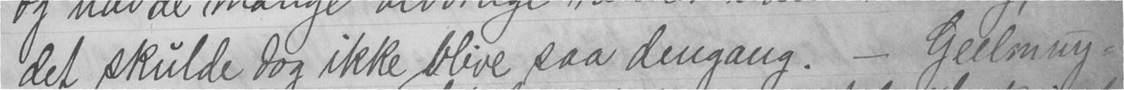det skulde dog ikke blive saa dengang. - Geelmuy-
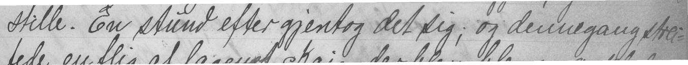stille. En stund efter gjentog det sig; og dennegang strei-
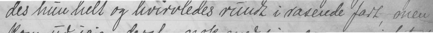des hun helt og hvirvledes rundt i rasende fart, men
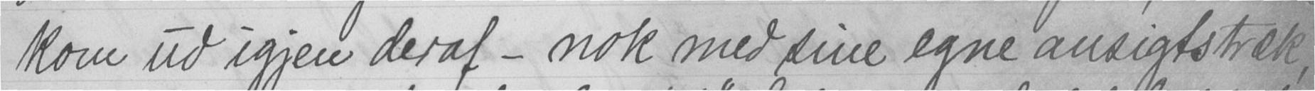kom ud igjen deraf - nok med sine egne ansigtstræk,
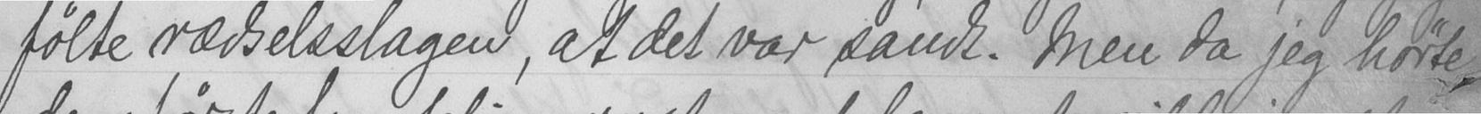følte rædselsslagen, at det var sandt. Men da jeg hørte
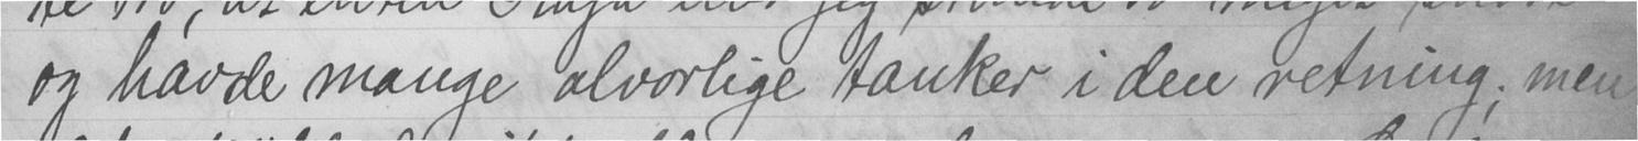og havde mange alvorlige tanker i den retning; men
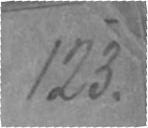123.
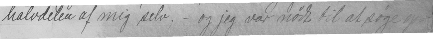halvdelen af mig selv, - og jeg var nødt til at søge op-
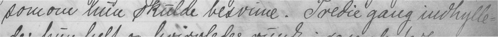som om hun skulde besvime. Tredie gang indhylle-
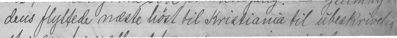dens flyttede næste høst til Kristiania til ubeskrivelig
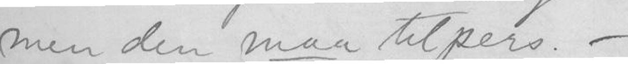men den maa tilpers. -
